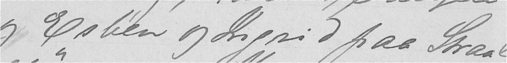og Esben og Ingrid paa Straal-
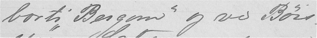"borti Bergom" og ved Børs-
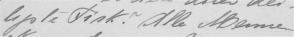ligste Fisk? Alle Menne-
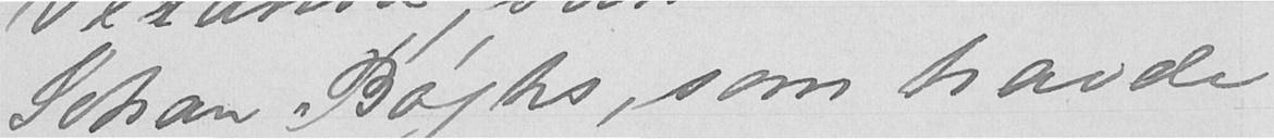Johan Bøghs, som havde
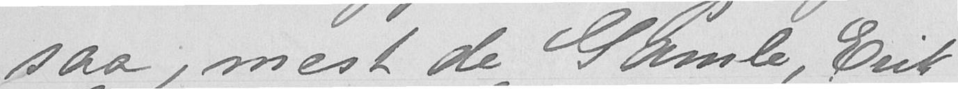saa, mest de Gamle, Erik
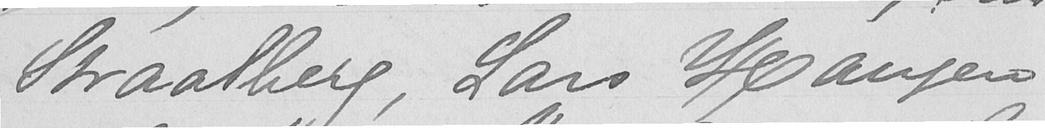Straalberg, Lars Haugen
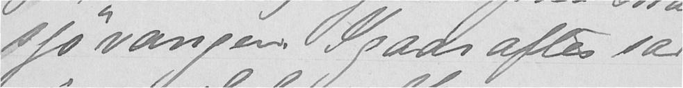sjøvangen. Igaar aftes sad
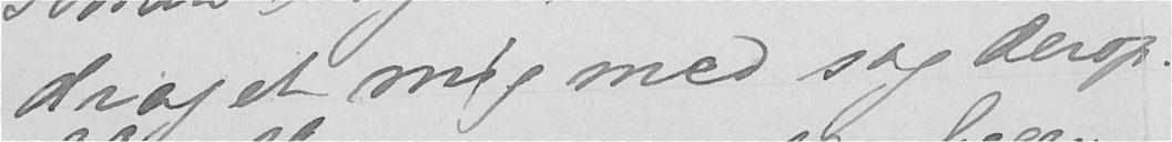draget mig med sig derop.
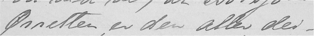Ørretten er den aller dei-
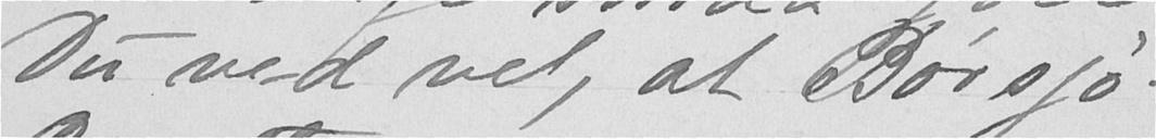Du ved vel, at Børsjø-
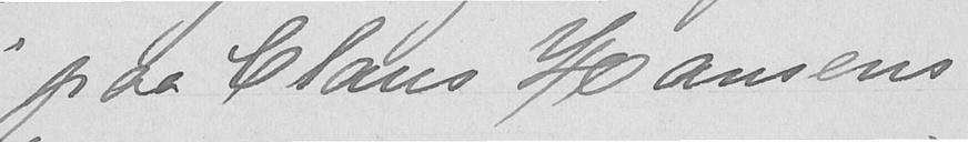jeg paa Claus Hansens
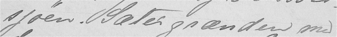sjøen. Sætergrænden med
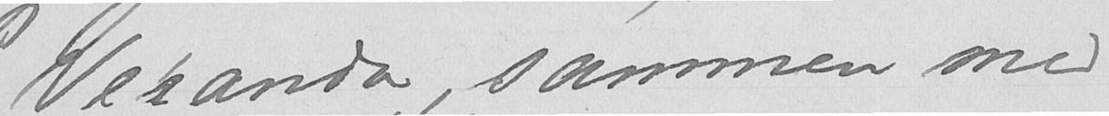Veranda, sammen med
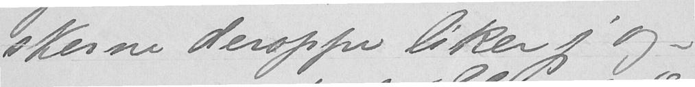skerne deroppe liker jeg og-
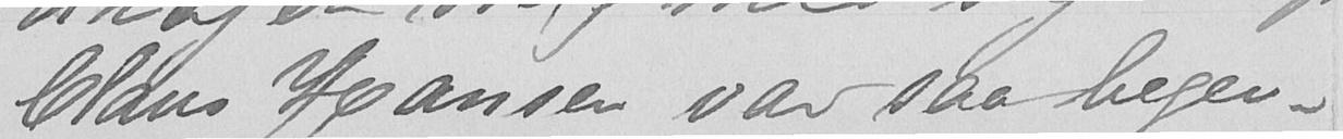Claus Hansen var saa begei-
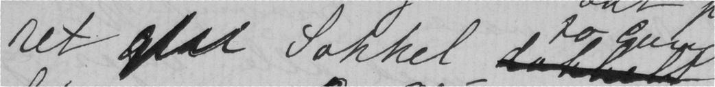ret glat Sokkel
